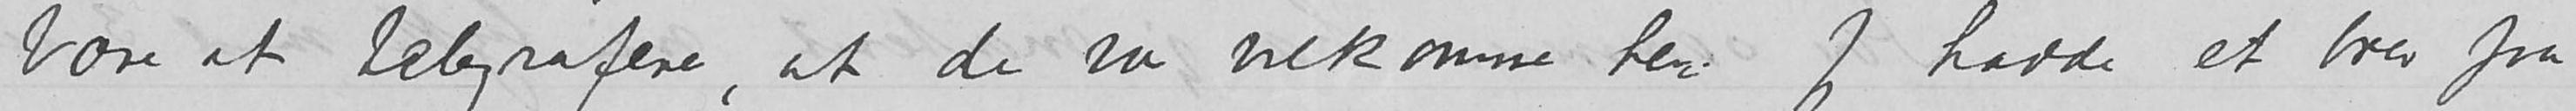bare at telegrafere, at de var velkomne her. Jeg hadde et brev fra
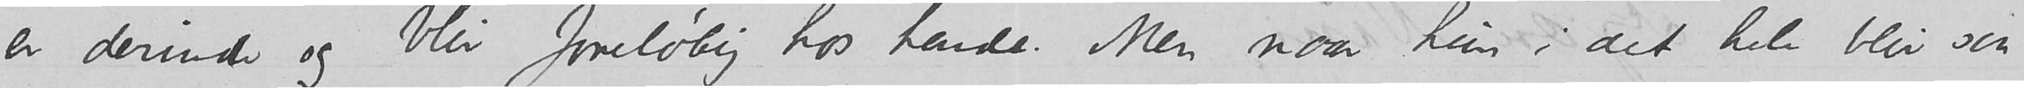er derinde og blir foreløbig hos hende. Men naar hun i det hele blir saa
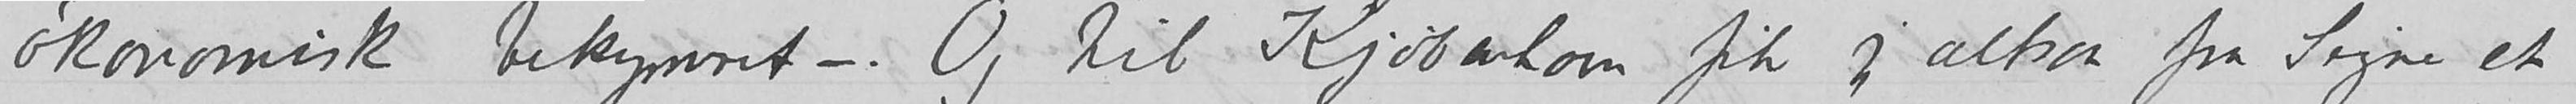økonomisk bekymret –. Og til Kjøbenhavn fik jeg altsaa fra Signe et
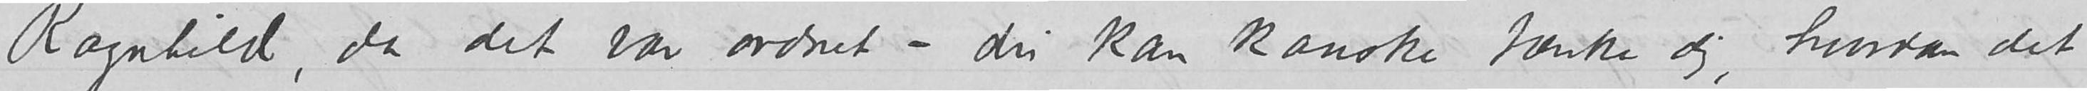Ragnhild, da det var ordnet – du kan kanske tænke dig, hvordan det
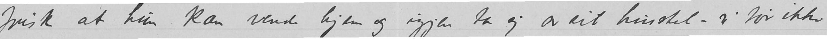frisk at hun kan vende hjem og igjen ta sig av sit husstel – jeg tør ikke
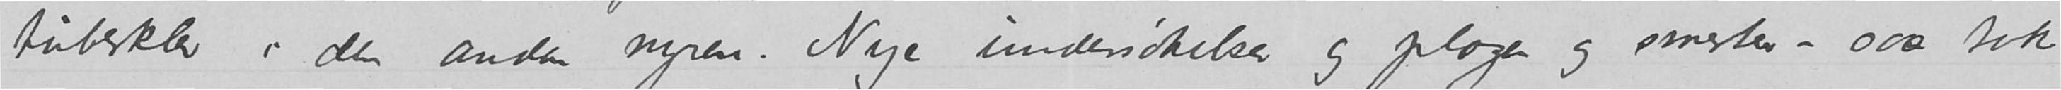tuberkler i den anden nyren. Nye undersøkelser og plager og smerter – saa tok
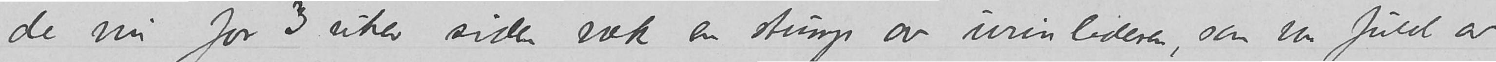de nu for 3 uker siden nok en stump av urinlederen, som var fuld av
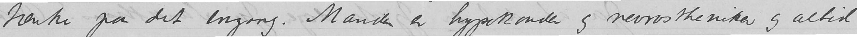tænke paa det engang. Manden er hypokonder og nevrostheniker og altid
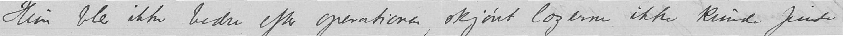Hun ble ikke bedre efter operationen, skjønt ægerne ikke kunde finde
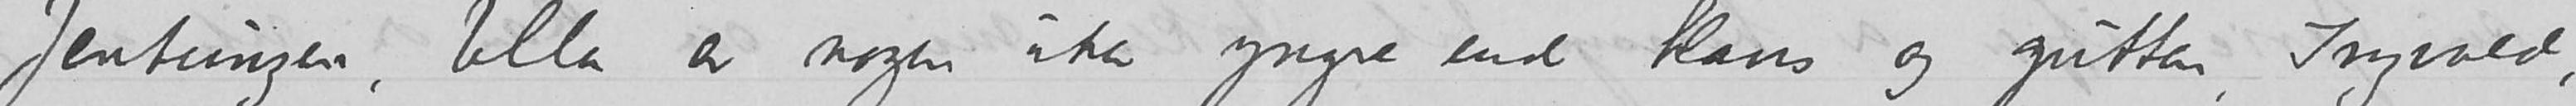Jentungen, Ulla er nogen uker yngre end Hans og gutten, Ingvald,
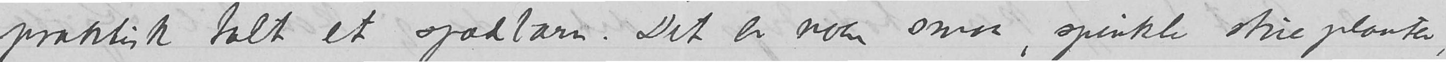praktisk talt et spædbarn. Det er noen smaa, spinkle stueplaner,
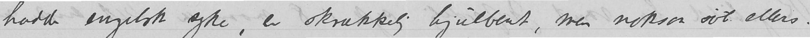hadde engelsk syke, er skrækkelig hjulbent, men noksaa søt ellers.
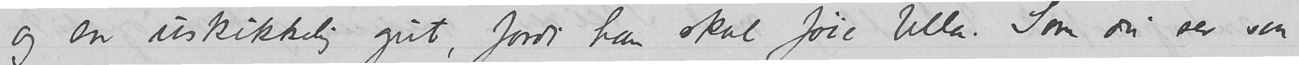og er uskikkelig gut, fordi han skal føie Ulla. Som du ser saa
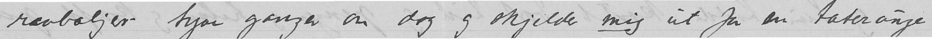rævbæljer tyve ganger om dag og skjelder mig ut for en taterunge
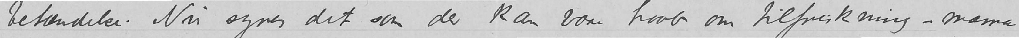betændelser. Nu synes det som der kan være haab om tilfriskning – mama
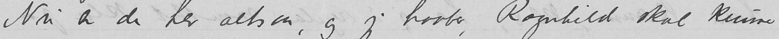Nu er de her altsaa, og jeg haaber, Ragnhild skal kunne
